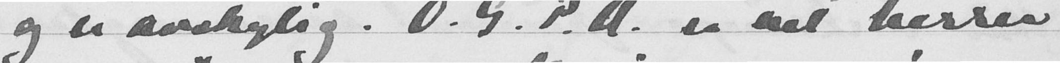og er avskylig. O. G. P. H. er vel herren
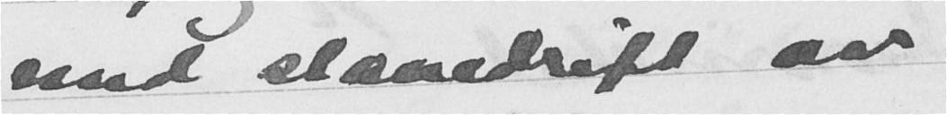med slavedrift av
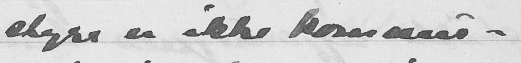styre er ikke kommu-
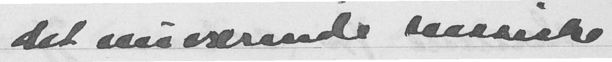det nuværende russiske
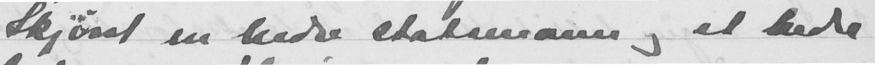Skjønt en bedre statsmann og et bedre
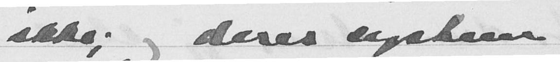ikke; og deres system
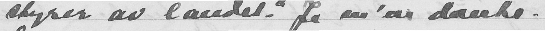styrer av landet? Je m'a danke.
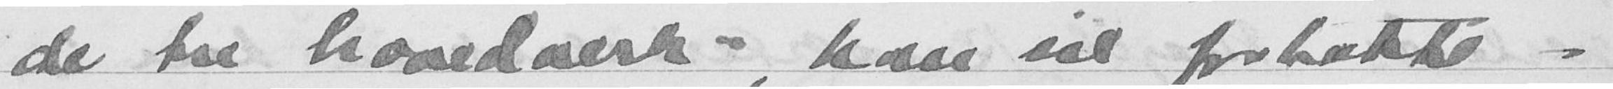de tre "hovedverk" han vil fortelle -
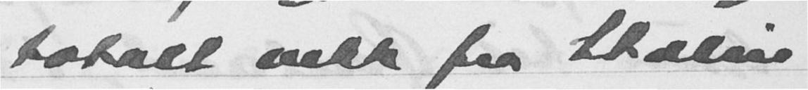totalt vekk fra Stalin
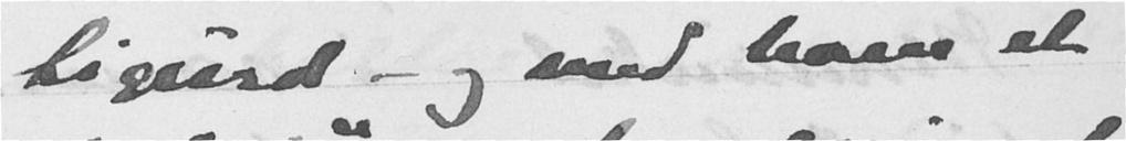Sigurd - og med ham et
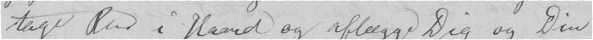tage Pen i Haand og aflægge Dig og Din
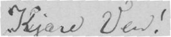Kjære Ven!
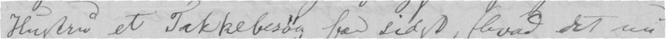Hustru et Takkebesøg for sidst, (hvad det nu
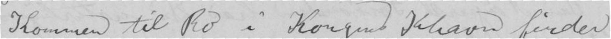Kommen til Ro i Kongens Khavn finder
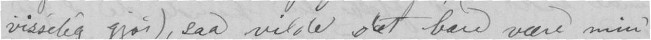visselig gjør), saa vilde det bare være min
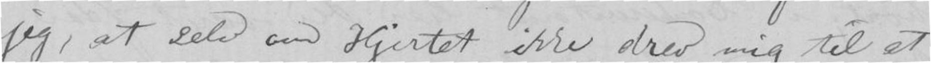jeg, at selv om Hjertet ikke drev mig til at
